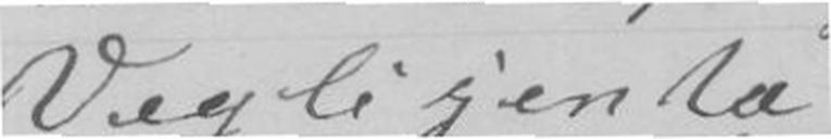Veglijenta
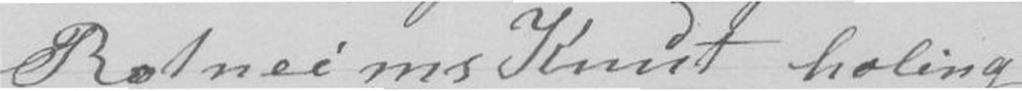Rotneims Knut haling
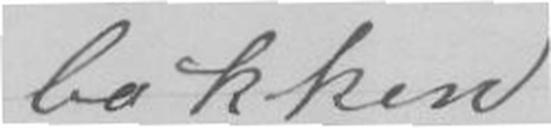bakken
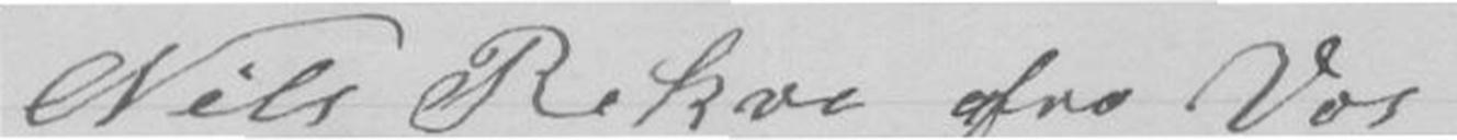Nils Rekve fra Vos
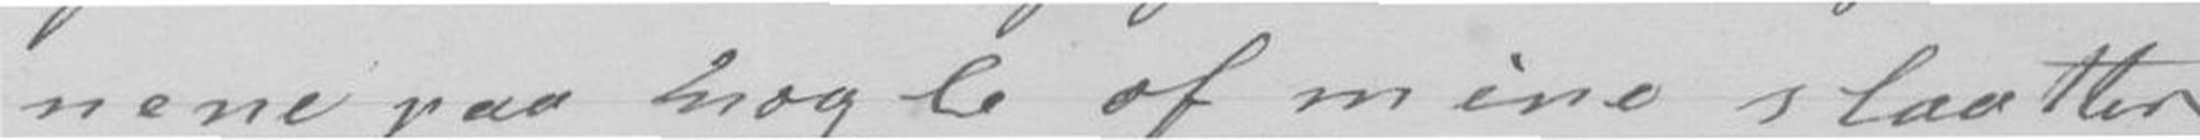nene paa nogle af mine slaatter
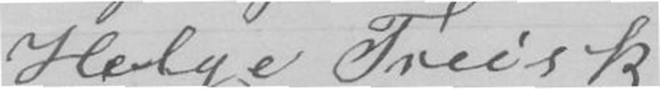Helge Treisk
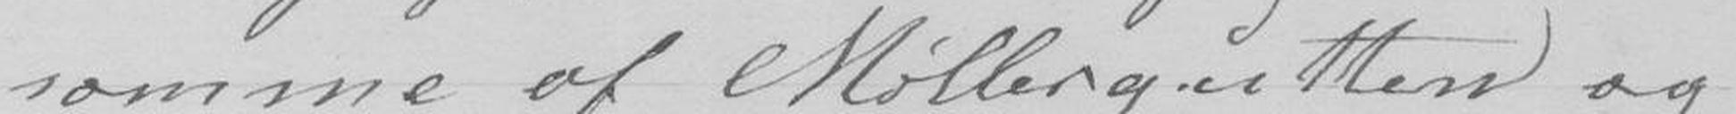somme af Møllergutten og
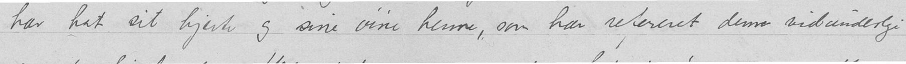har hat sit hjerte og sine øine henne, som har refereret denne vidunderlige
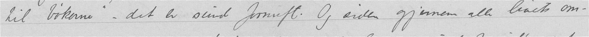til bøkerne" – det er sund fornuft. Og siden gjennem alle livets om-
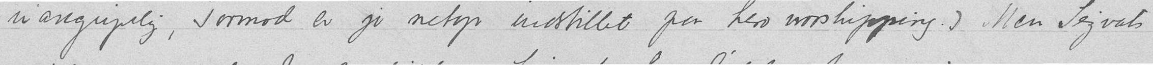uangripelig, Tormod er jo netop indstillet paa hero worshipping.) Men Sigvats
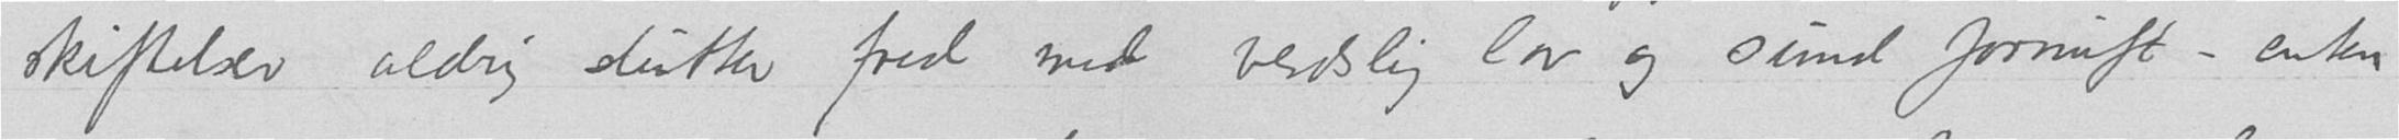skiftelser aldrig slutter fred med verdslig lov og sund fornuft – enten
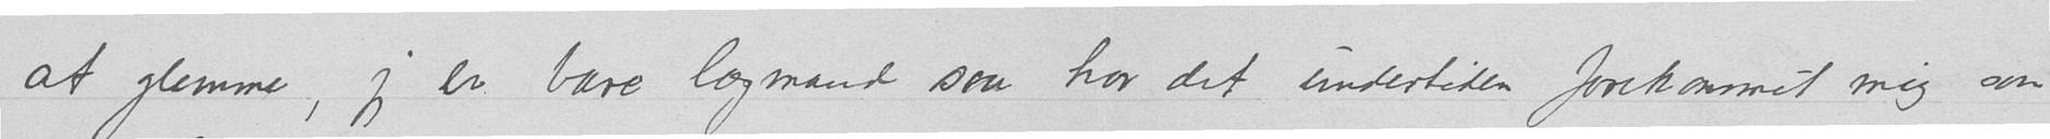at glemme, jeg er bare lægmand saa har det undertiden forekommet mig som
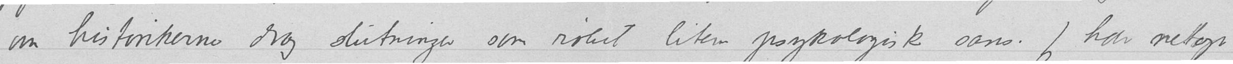om historikerne drog slutninger som røbet liten psykologisk sans. Jeg har netop
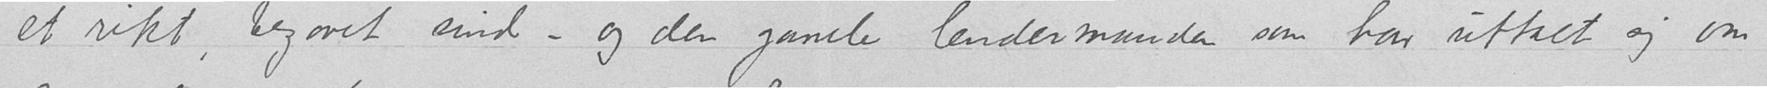et rikt, begavet sind – og den gamle lendermanden som har uttalt sig om
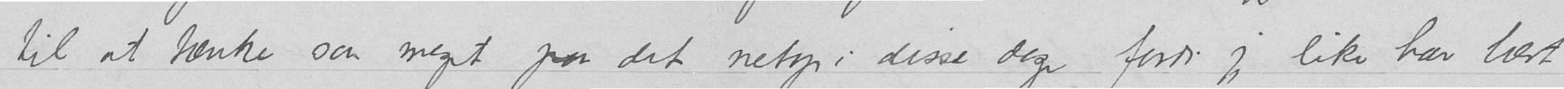til at tænke saa meget paa det netop i disse dage fordi jeg like har læst
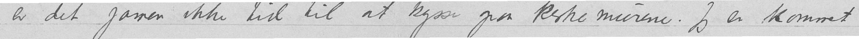er det jamen ikke tid til at kysse paa kirkemurene. Jeg er kommet
